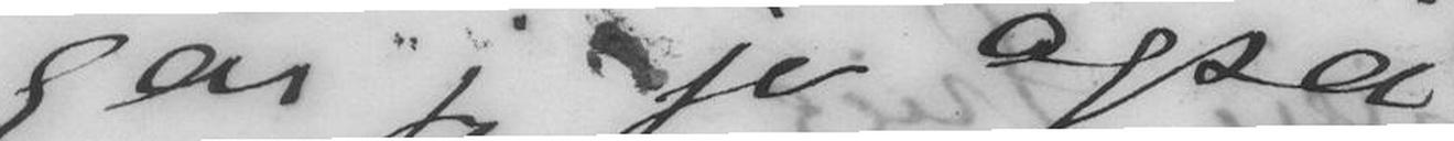går jeg jo også
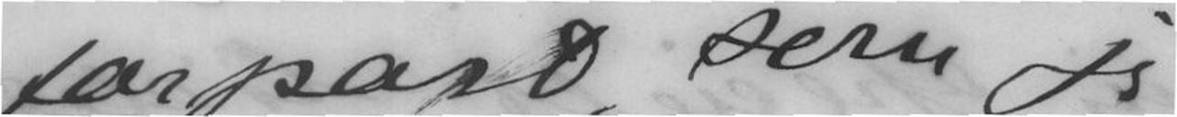forpart som jeg -
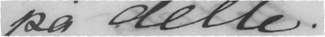på dette.
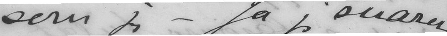som jeg - Ja jeg svarer
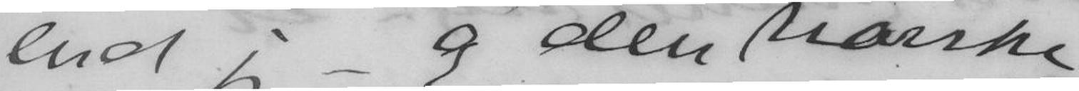end jeg - og den norske
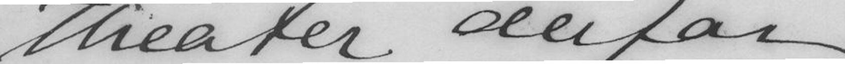theater derfor
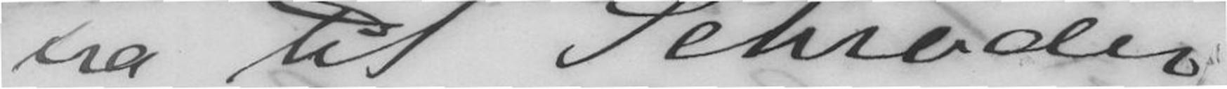fra til Schrøder
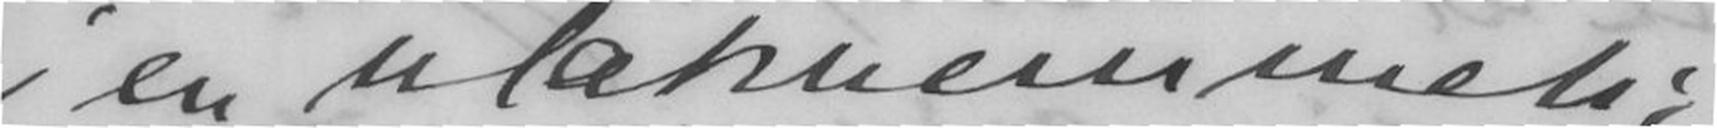i en utaknemmelig
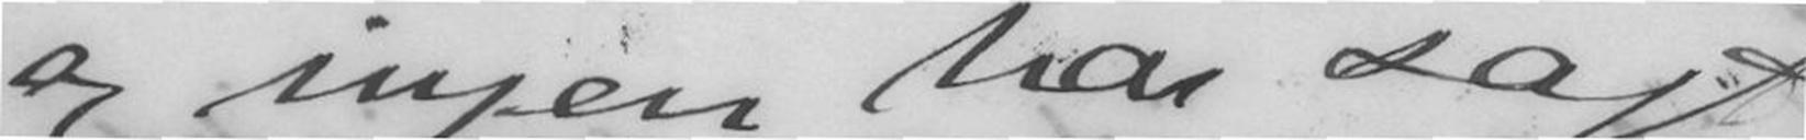og ingen har sagt
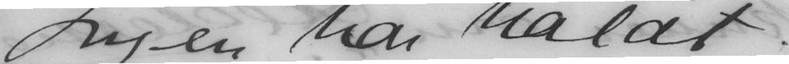Ingen har holdt
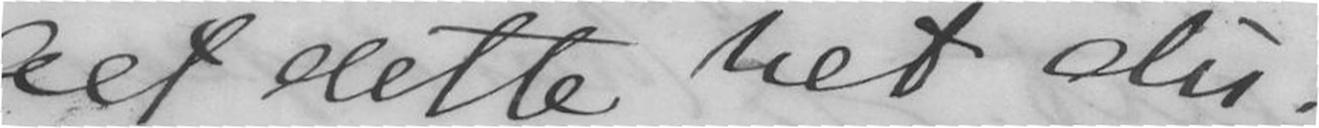alt dette vet du -
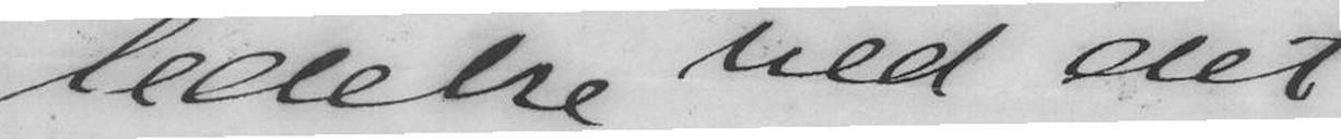ledelse ved det
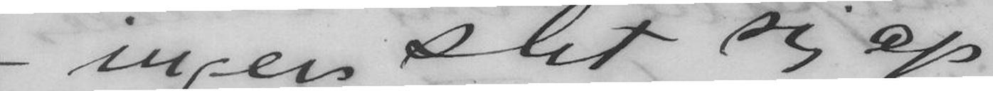- ingen slet sig op
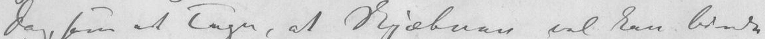Dog, som et Tegn, at Skjæbnen vel kan binde
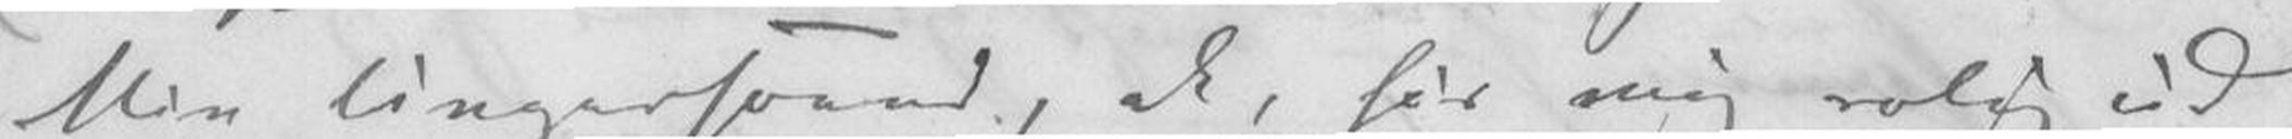Min Ungersaand, ak, hør mig rolig ud,
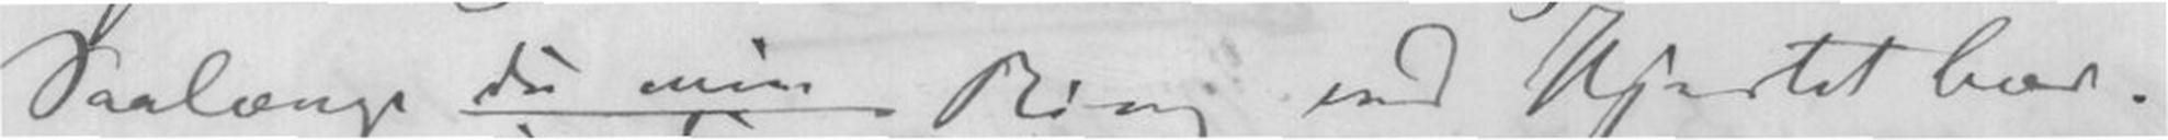Saalænge du min Ring end Hjertet var.
